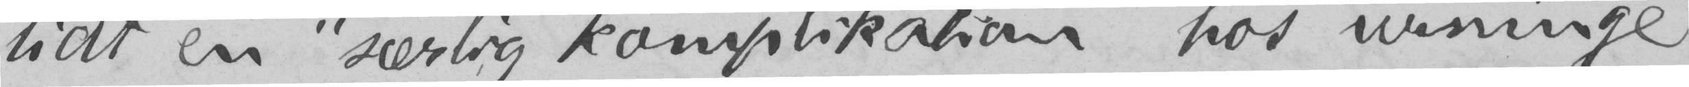lidt en «særlig komplikation» hos urninge
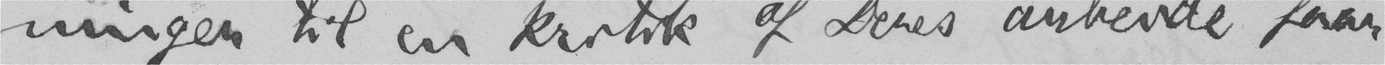ninger til en kritik af Deres arbeide faar
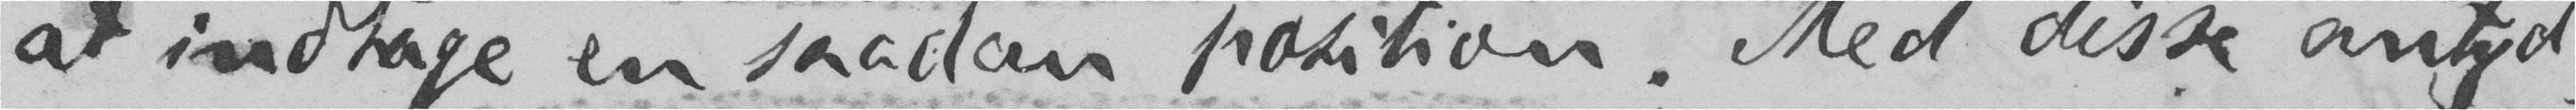at indtage en saadan posistion. Med disse antyd-
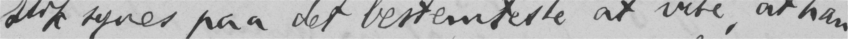stik synes paa det bestemteste at vise, at han
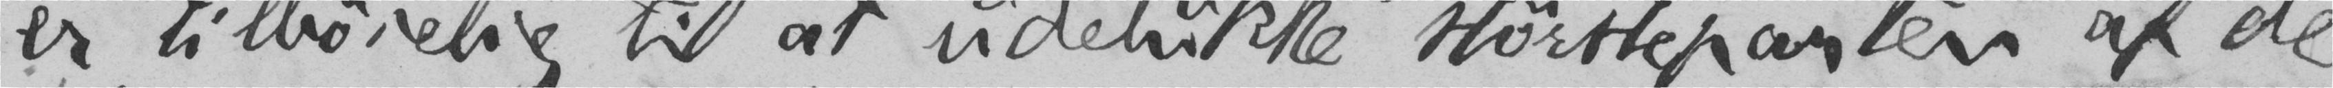er tilbøielig til at udelukke størsteparten af de
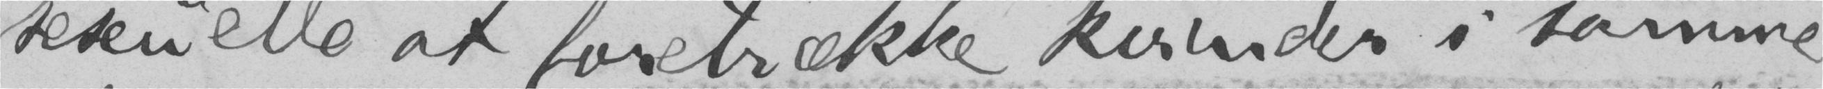seksuelle at foretrække kvinder i samme
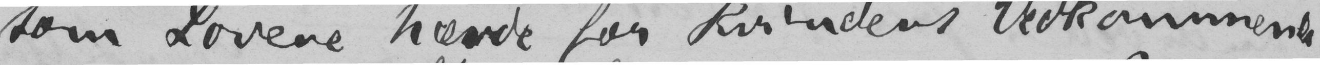som Lovene hævde for Kvindens Vedkommende,
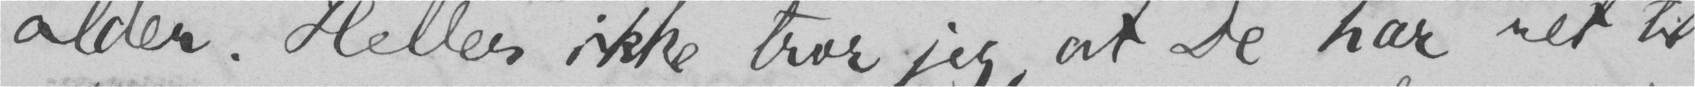alder. Heller ikke tror jeg, at De har ret til
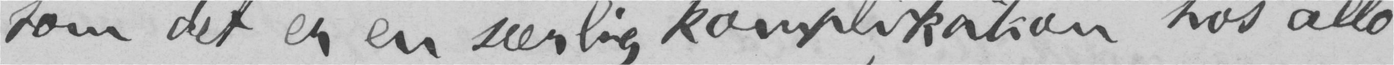som det er en særlig komplikation hos allo-
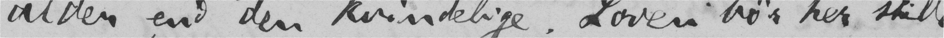alder end den kvindelige. Loven bør her stille
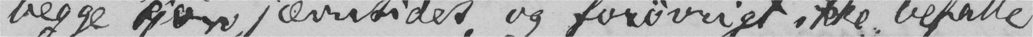begge kjøn jævnsides, og forøvrigt ikke befatte
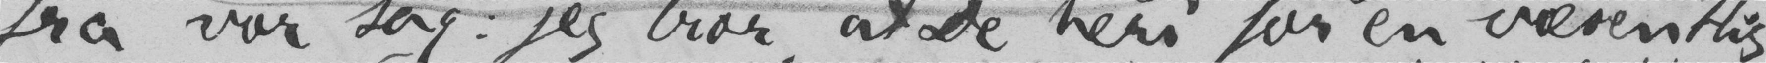fra vor sag: jeg tror, at De heri for en væsentlig
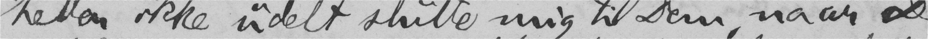heller ikke udelt slutte mig til Dem, naar De
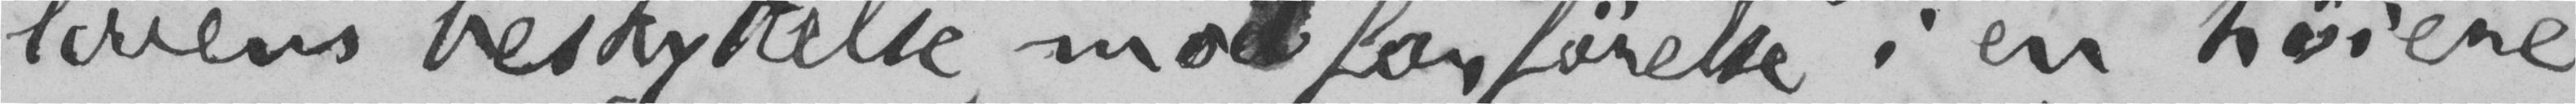lovens beskyttelse mod forførelse i en høiere
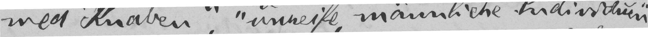med «Knaben», «unreife männliche Individuen»
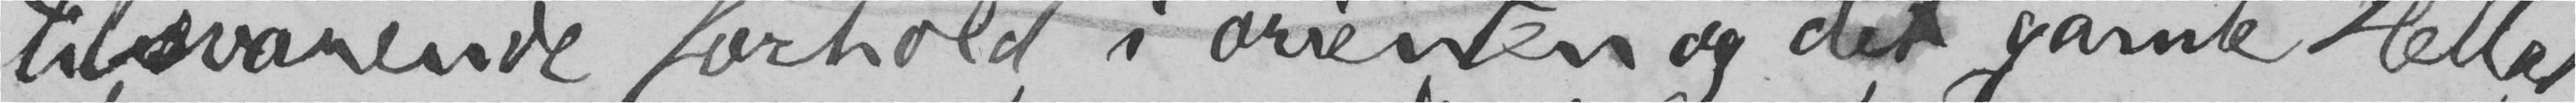tilsvarende forhold i orienten og det gamle Hellas
